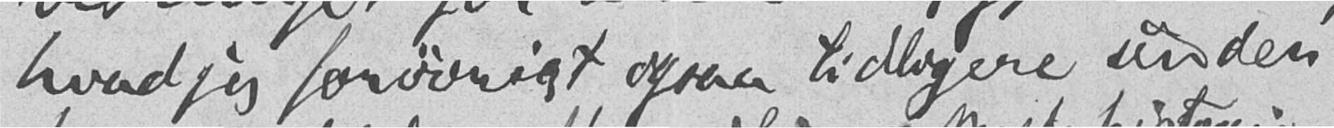hvad jeg forøvrigt ogsaa tidligere under
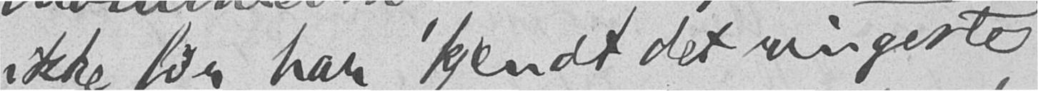ikke før har kjendt det ringeste
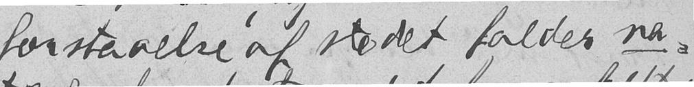forstaaelse af stedet falder na-
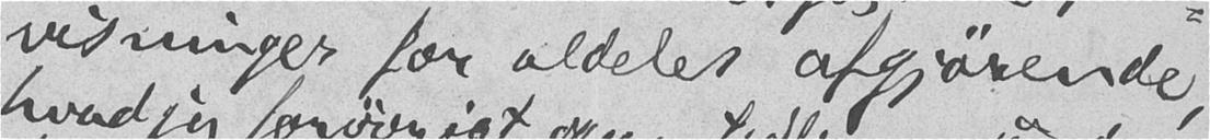visninger for aldeles afgjørende,
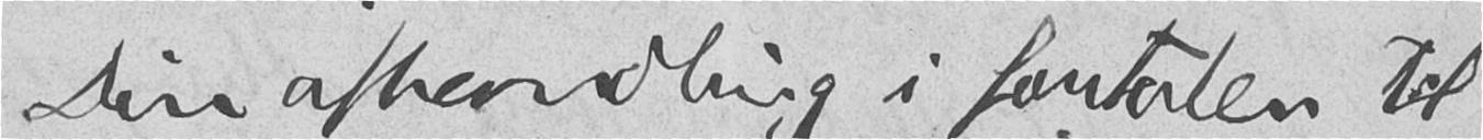Din afhandling i fortalen til
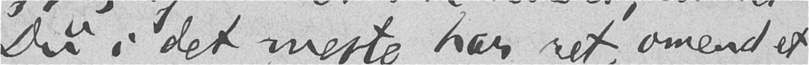Du i det meste har ret, omend et
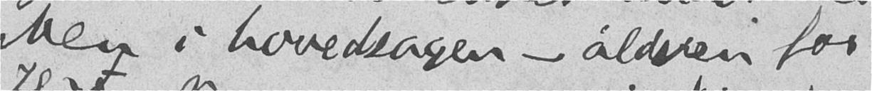Men i hovedsagen - alderen for
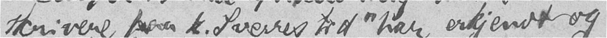skrivere paa k. Sverres tid" har erkjendt og
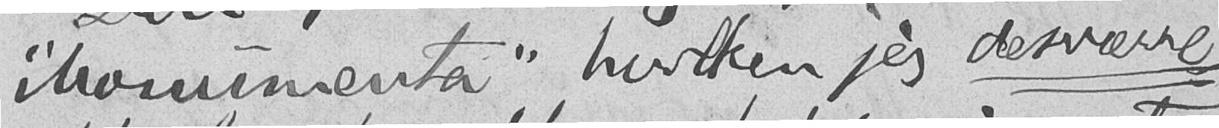"Monumenta", hvilken jeg desværre
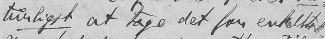turligst at tage det for enkelttal.
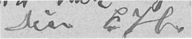Din EH.
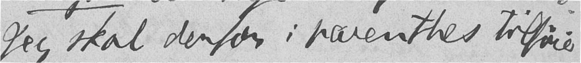Jeg skal derfor i parenthes tilføie
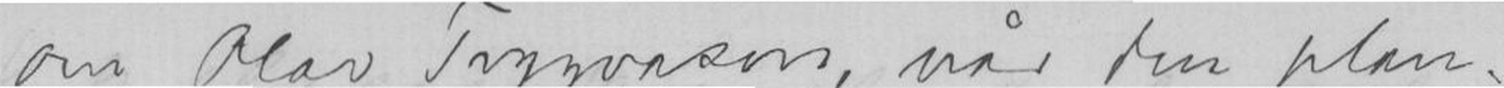om Olav Trygvason, når den plan
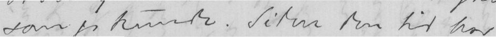som jeg kunde. Siden den tid har
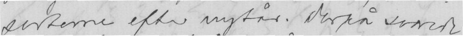serterne efter nytår. Derpå svarede
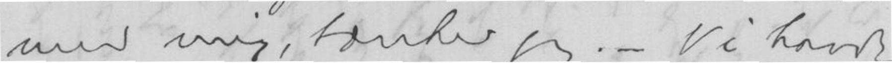med mig, tænker jeg.- Vi havde
